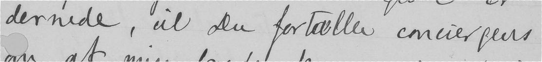dernede, vil Du fortælle conciergens
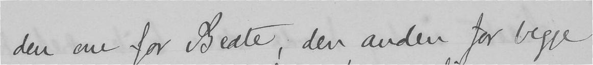den ene for Beate, den anden for begge
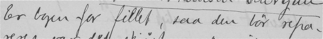Er bogen for fillet, saa den bør repa-
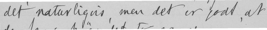det naturligvis, men det er godt, at
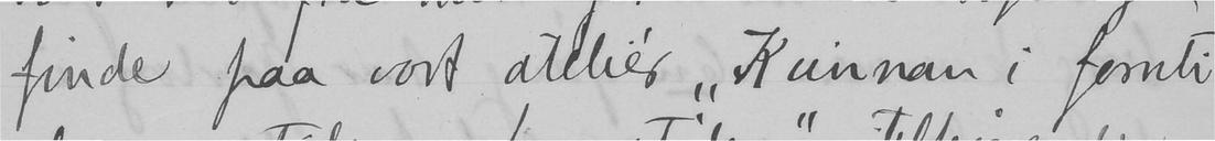finde paa vort atélier "Kvinnan i fornti
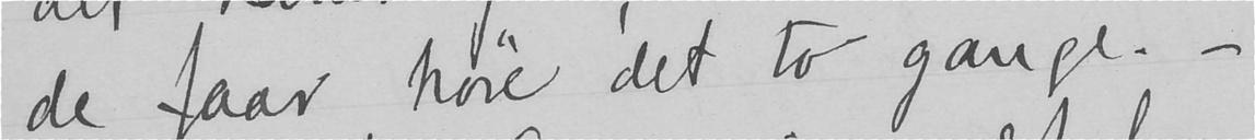de faar høre det to gange. -
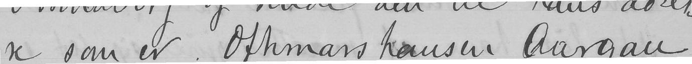se som er Othmars hausen Aurgau
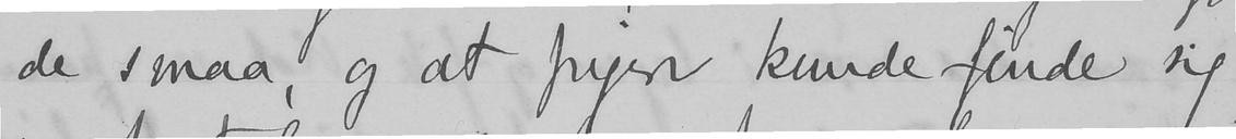de smaa, og at pigen kunde finde sig
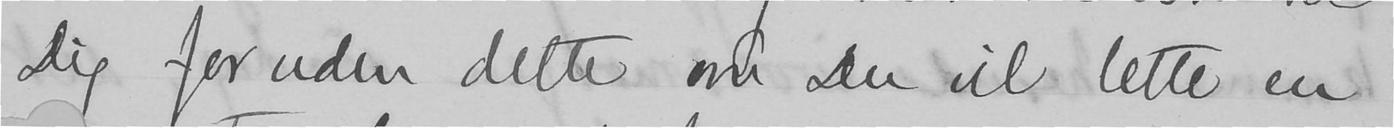Dig foruden dette om Du vil lette en
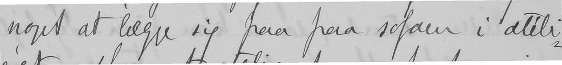noget at lægge sig paa paa sofaen i atéli-
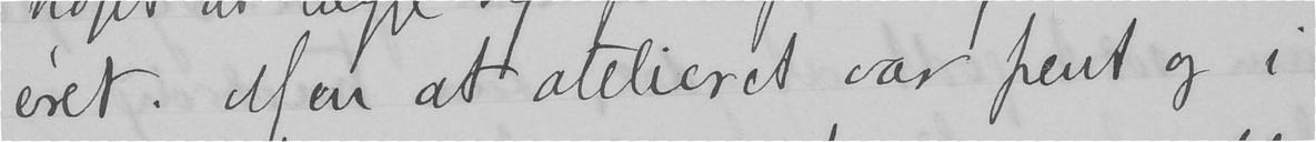eret. Men at atelieret var pent og i
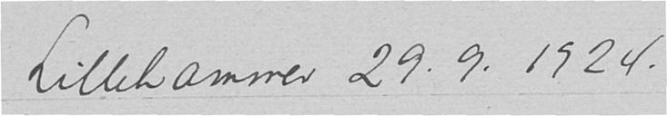Lillehammer 29.9.1924.
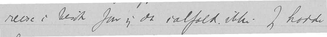reise i besøk faar jeg da ialfald ikke. Jeg hadde
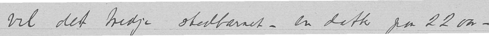vel det tredje stedbarnet - en datter paa 22 aar -
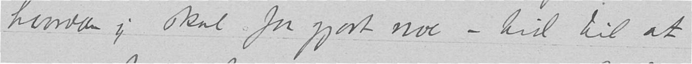hvordan jeg skal faa gjort noe – tid til at
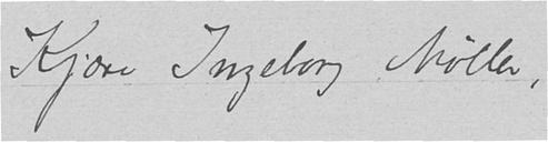Kjære Ingeborg Møller,
